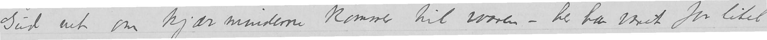Gud vet om kjærminderne kommer til vaaren - her har været for litet
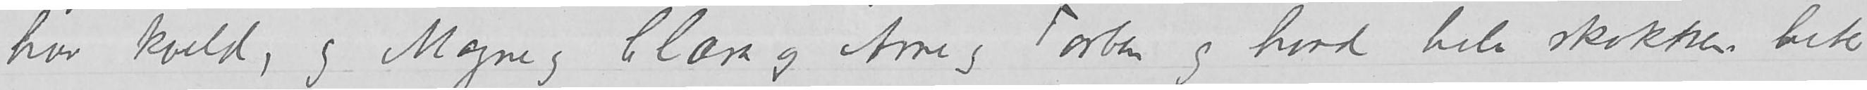hver kveld, og Magne og Clara og Arne og Torben og hvad hele skokken heter
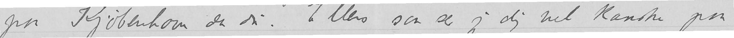paa Kjøbenhavn da du.  Ellers saa ser jeg dig vel kanske paa
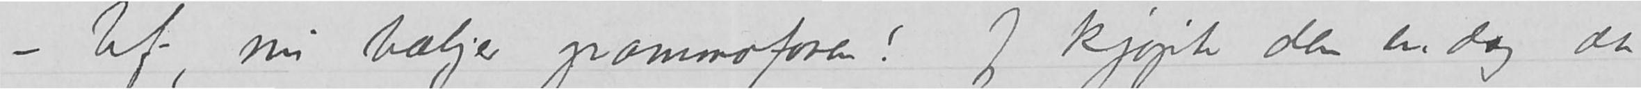- Uf, nu bæljer grammofonen! Jeg kjøpte den en dag da
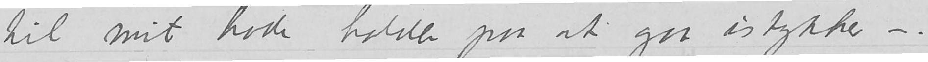til mit hode holder paa at gaa istykker -
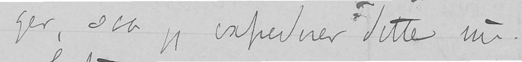ger, saa jeg expederer dette nu.
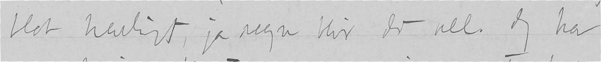blot hærligt, jo regn blir det vel. Jeg har
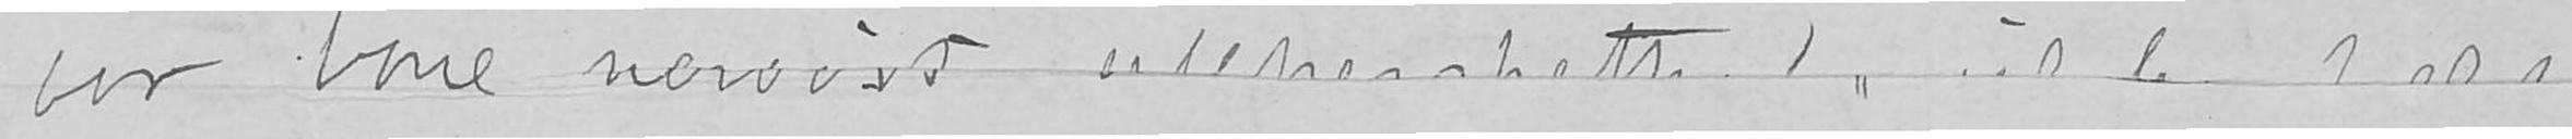var bare nervøst utehershett, ud lan hoa
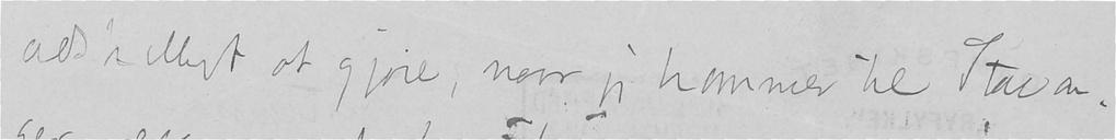adskilligt at gjøre, naar jeg kommer til Stavan-
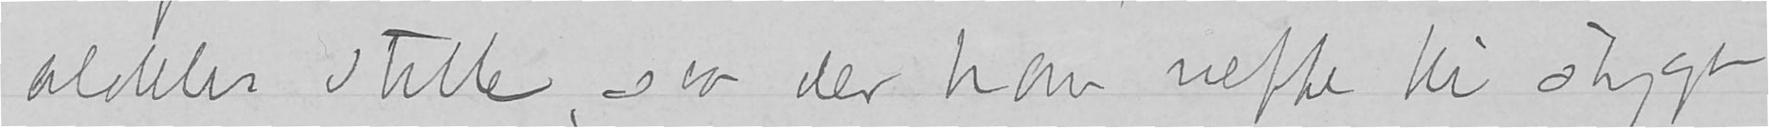aldeles stille, saa der kan neppe bli stygt
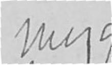myg
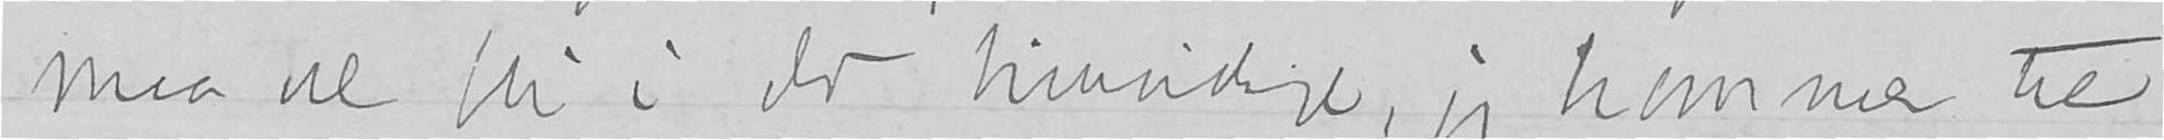maa vel bli i det hinsidige, jeg kommer til
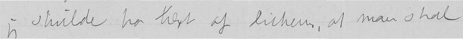jeg skulde ha tænt af lichem, at man skal
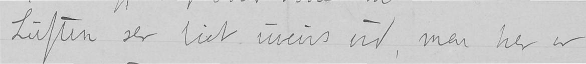Luften ser lidt uveirs ud, men her er
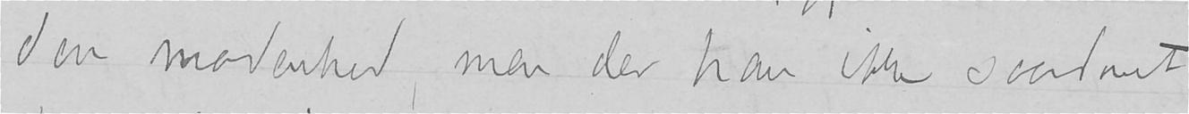den modenhed, men der kan ikke saadant
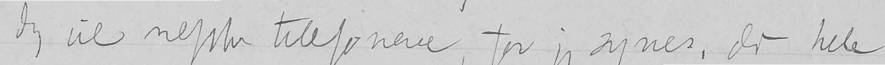Jeg vil neppe telefonere, for jeg synes, det hele
